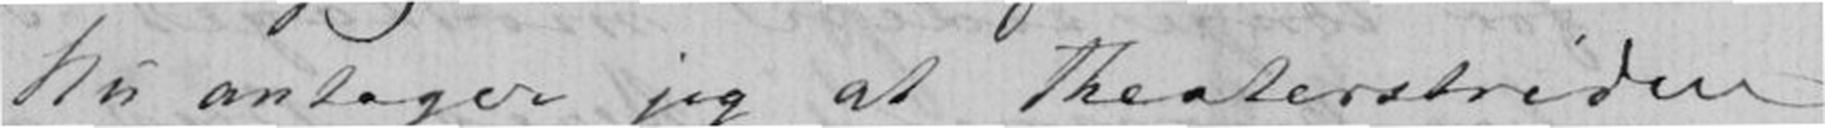Nu antager jeg at Theaterstriden
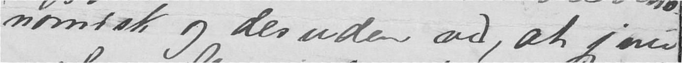nomisk og desuden ved, at jeg nu
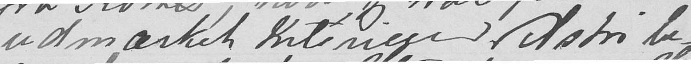udmærket Intérieur. Astri be-
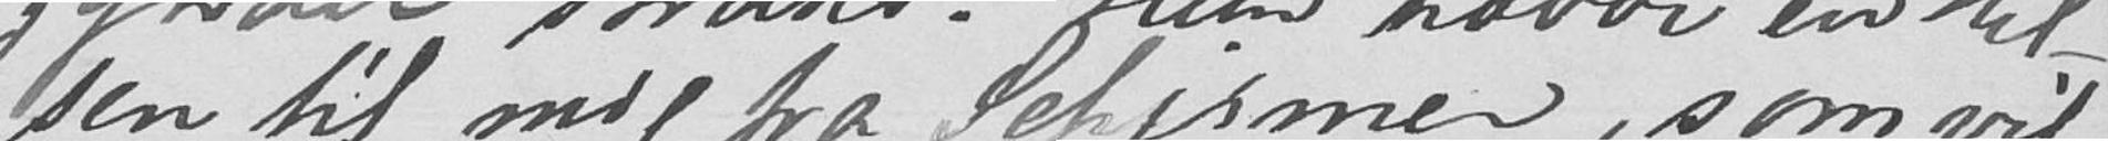sen til mig fra Schirmer, som vil-
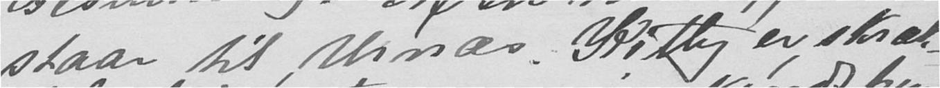staar til, Urnæs. Kitty er skræk-
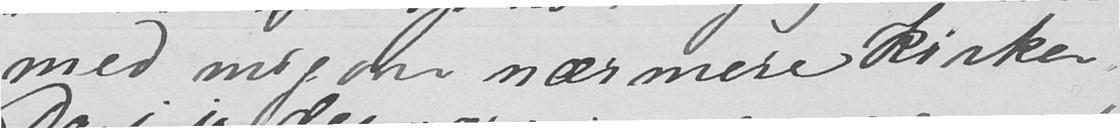med mig om nærmere Kirker.
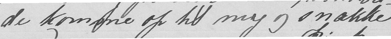de komme op til mig og snakke
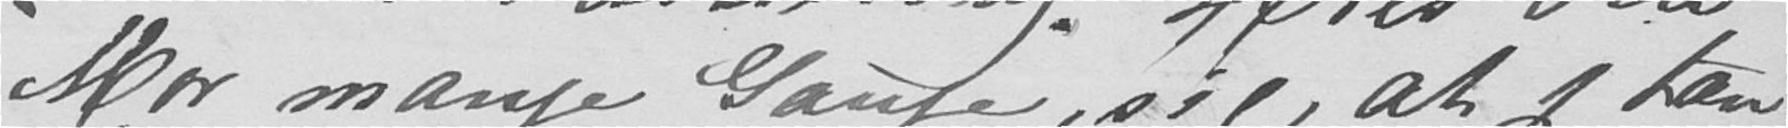Mor mange Gange, sig, at jeg tæn-
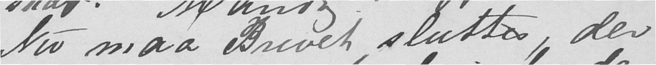Nu maa Brevet sluttes, der
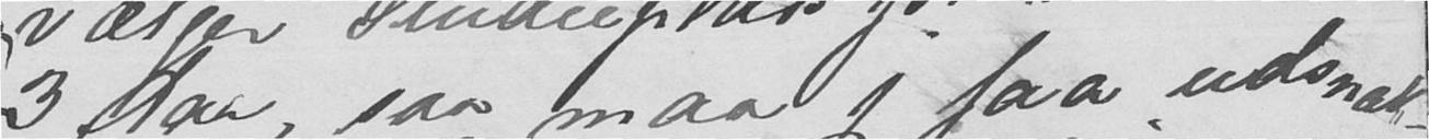3 Aar, saa maa jeg faa udsnak-
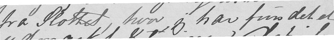fra Slottet, hvor jeg har fundet et
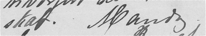skab. Mandag.
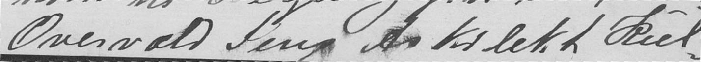Overvæld Jens Arkilekt Kiel-
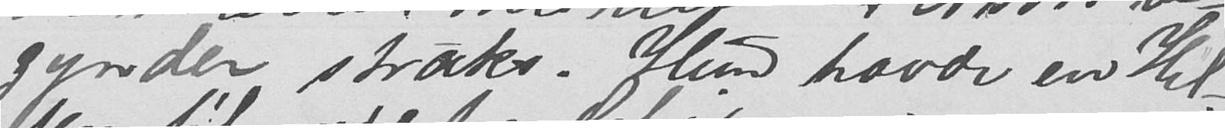gynder straks. Hun havde en Hil-
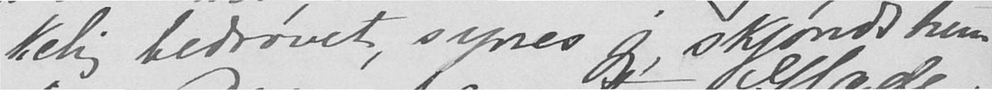kelig bedrøvet, synes jeg, skjøndt hun
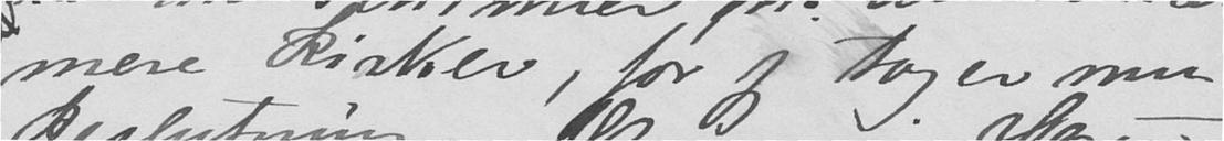mere Kirker, før jeg tager min
